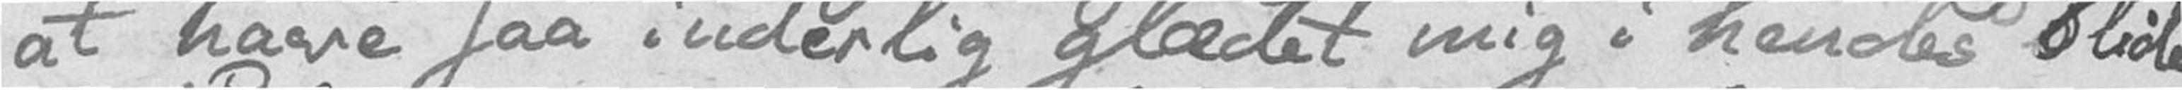at have saa inderlig glædet mig i hendes blide
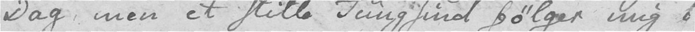Dag, men et stille Tungsind følger mig i
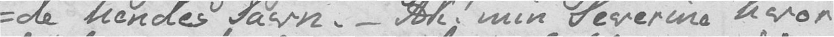-de hendes Savn. – Ak! min Severine hvor
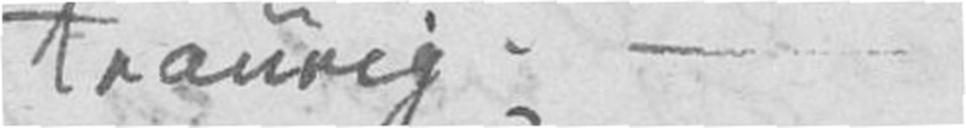traurig. –
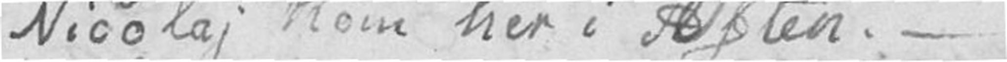Nicolaj kom her i Aften. –
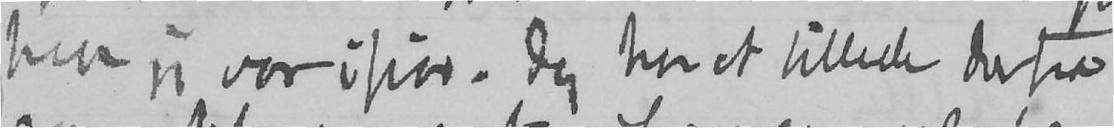hvor jeg var ifjor. Jeg har et billede derfra
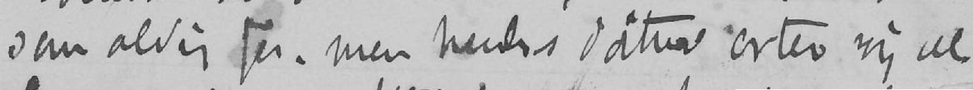som aldrig før. Men hendes døttre arter sig vel.
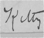Kitty
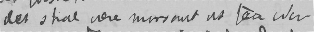Det skal være morsomt at faa eder
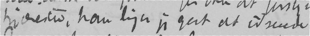kjæresten, han liger jeg godt at udsende.
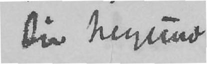Din hengivne
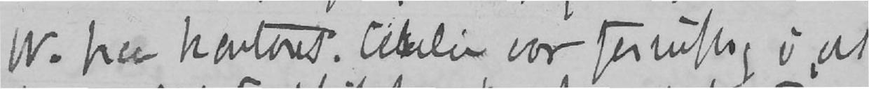W. paa kontoret. Cecilie var fornuftig i, at
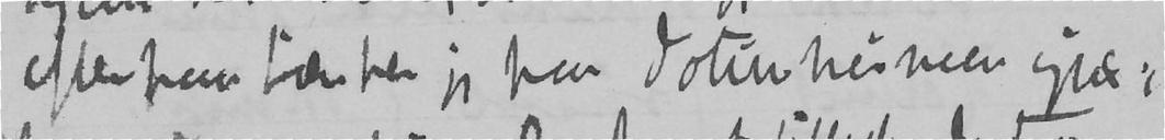efterpaa tænker jeg paa Jotunheimen igjen,
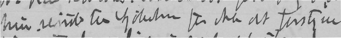hun reiste til Kjøbenhavn for ikke at forstyrre
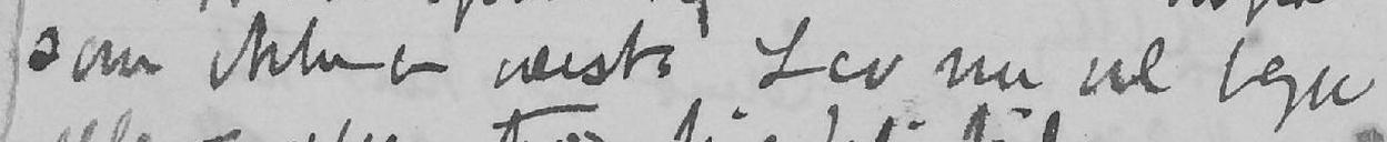som ikke er værst. Lev nu vel begge
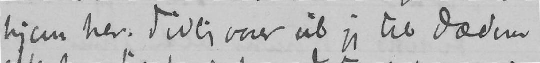hjem her. Tidlig vaar vil jeg til Jæderen
